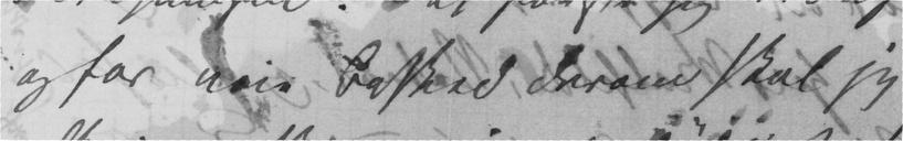og har nøie Besked derom skal jeg
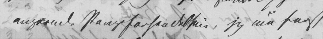angaende Pengeforsendelsen, jeg må først
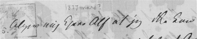Tilgiv mig kjere Alf at jeg ikke kan
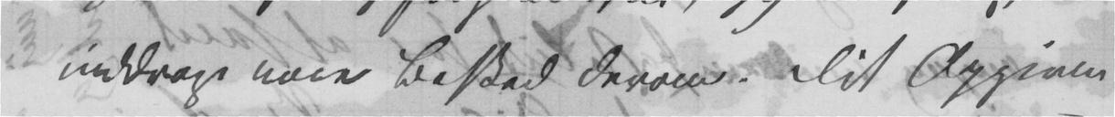inddrage nøie Besked derom. Dit Opgiven
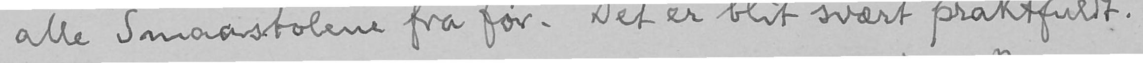alle Smaastolene fra før. Det er blit svært praktfuldt.
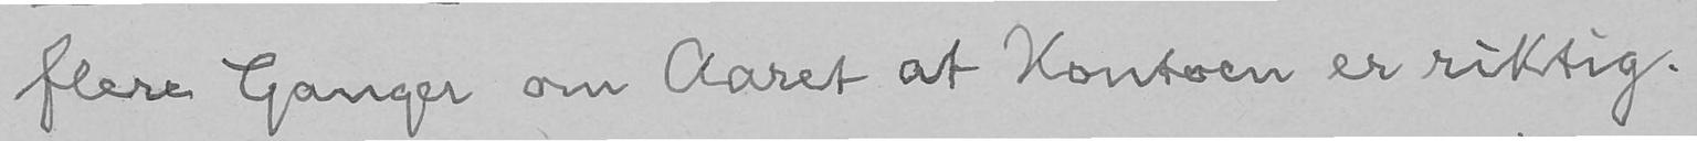flere Ganger om Aaret at Kontoen er riktig.
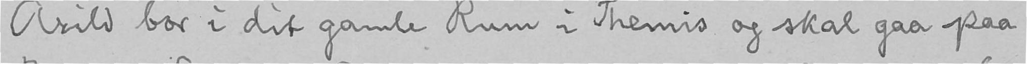Arild bor i dit gamle Rum i Themis og skal gaa paa
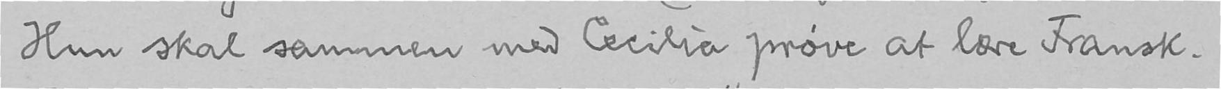Hun skal sammen med Cecilia prøve at lære Fransk.
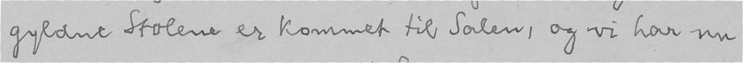gyldne Stolene er kommet til Salen, og vi har nu
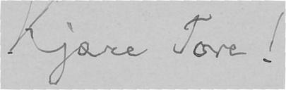Kjære Tore!
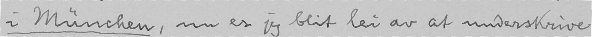i München, nu er jeg blit lei av at underskrive
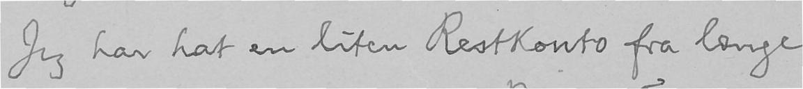Jeg har hat en liten Restkonto fra længe
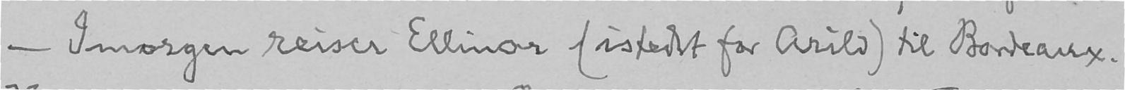- Imorgen reiser Ellinor (istedet for Arild) til Bordeaux.
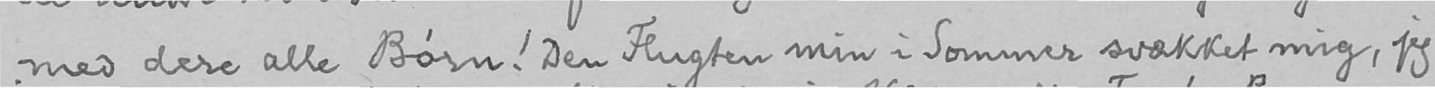med dere alle Børn! Den Flugten min i Sommer svækket mig, jeg
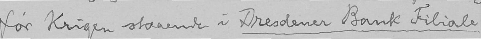før Krigen staaende i Dresdener Bank Filiale
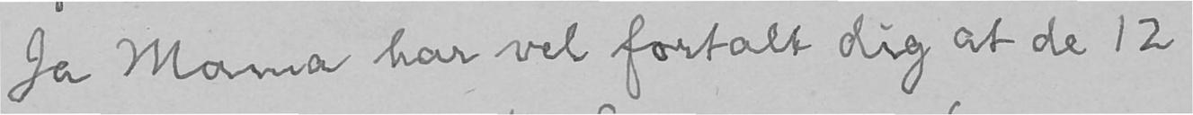Ja Mama har vel fortalt dig at de 12
